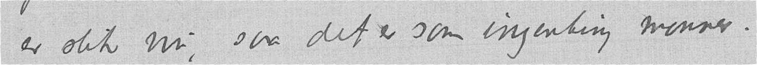er slik nu, saa det er som ingenting monner.
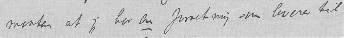maaten at jeg har en forretning som leverer til
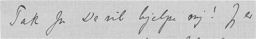Tak for De vil hjelpe mig! Jeg er
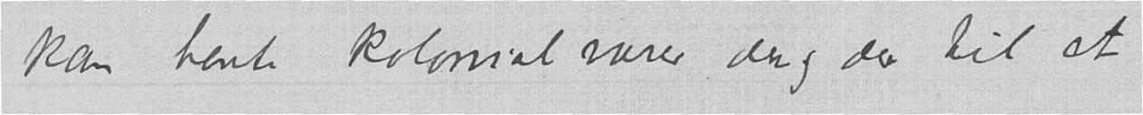kan hente kolonialvarer, der og der til et
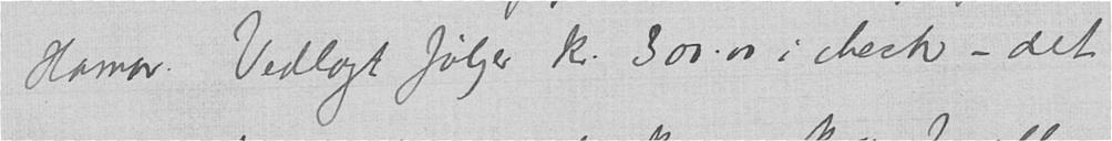Hamar. Vedlagt følger kr. 300.00 i check – det
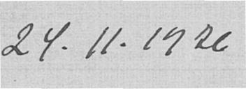24.11.1926
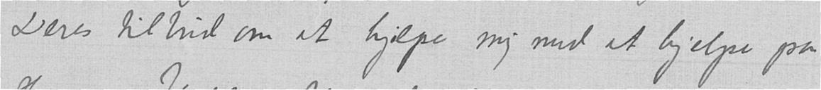Deres tilbud om at hjelpe mig med at hjelpe paa
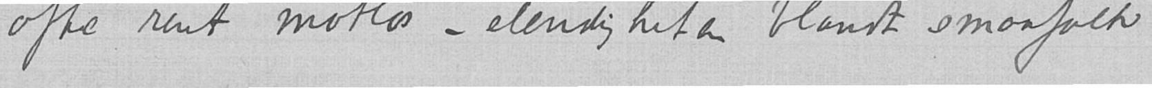ofte rent motløs – elendigheten blandt smaafolk
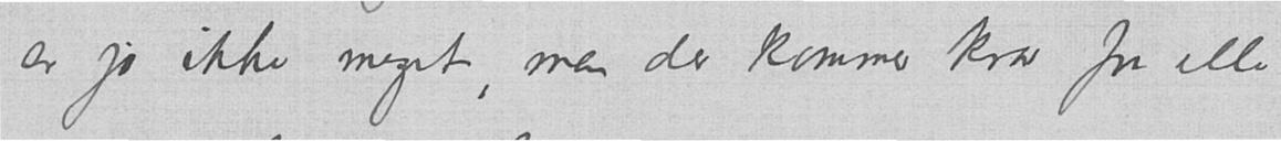er jo ikke meget, men der kommer krav fra alle
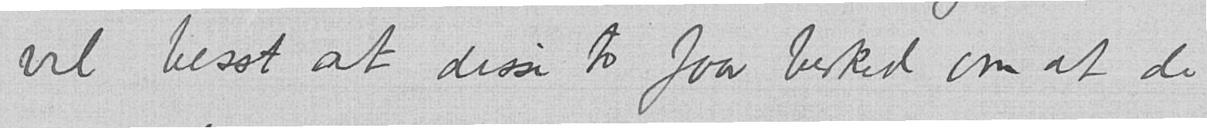vel besst at disse to faa besked om at de
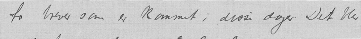to brever som er kommet i disse dager. Det blir
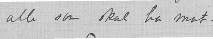alle som skal ha mat.
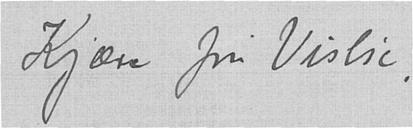Kjære fru Vislie,
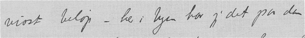visst beløp – her i byen har jeg det paa den
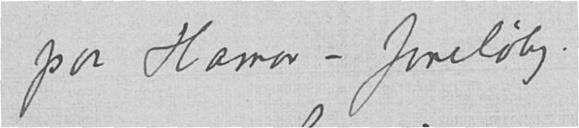paa Hamar – foreløbig.
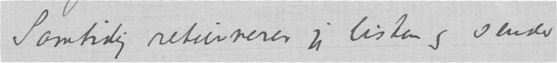Samtidig returnerer jeg listen og sender
